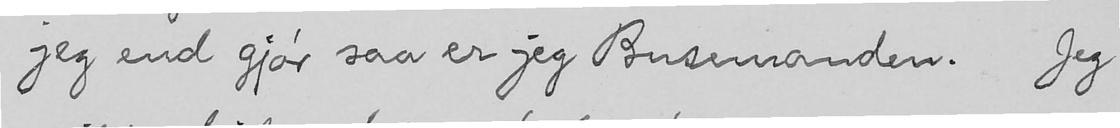jeg end gjør saa er jeg Busemanden. Jeg
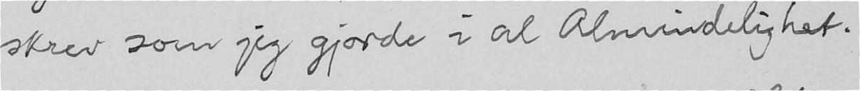skrev som jeg gjorde i al Almindelighet.
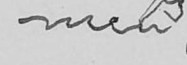men
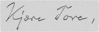Kjære Tore,
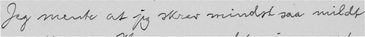Jeg mente at jeg skrev mindst saa mildt
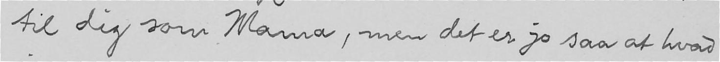til dig som Mama, men det er jo saa at hvad
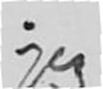jeg
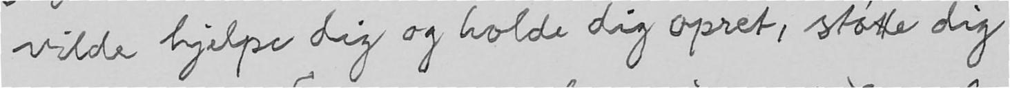vilde hjelpe dig og holde dig opret, støtte dig
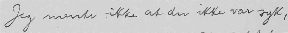Jeg mente ikke at du ikke var syk,
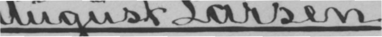August Larsen,
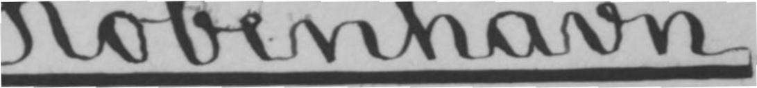København.
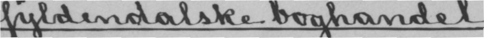Gyldendalske boghandel,
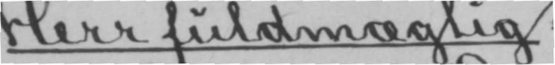Herr fuldmægtig
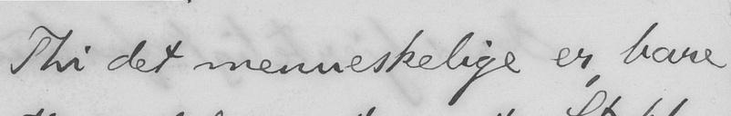Thi det menneskelige er, bare
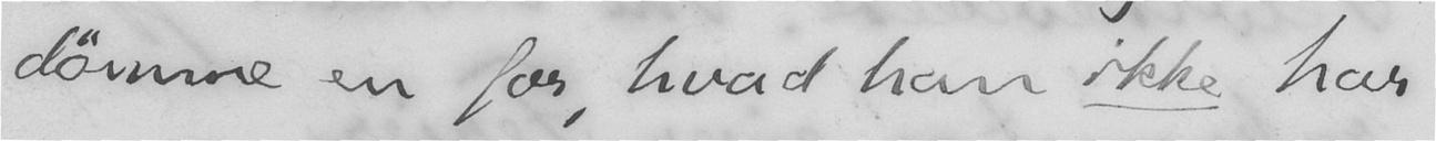dømme en for, hvad han ikke har
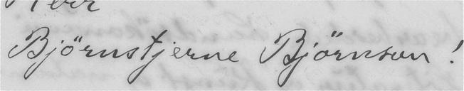Bjørnstjerne Bjørnson!
